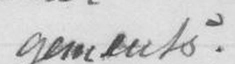gement.
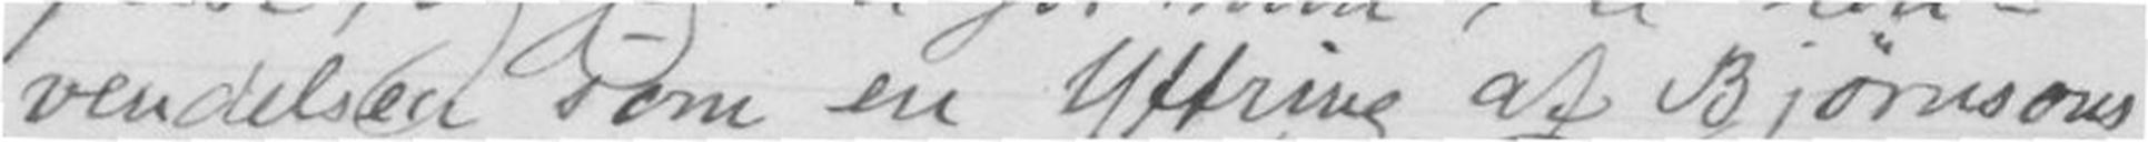vendelsen som en Yttring af Bjørnsons
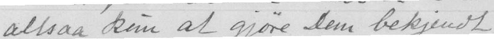altsaa kun at gjøre Dem bekjendt
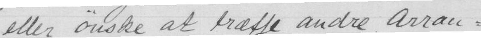eller ønske at træffe andre Arran-
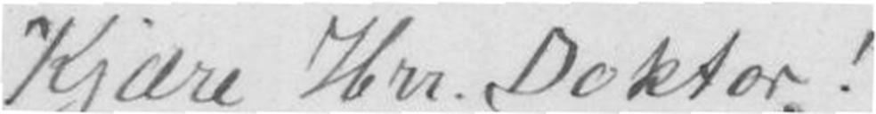Kjære Hrr. Doktor!
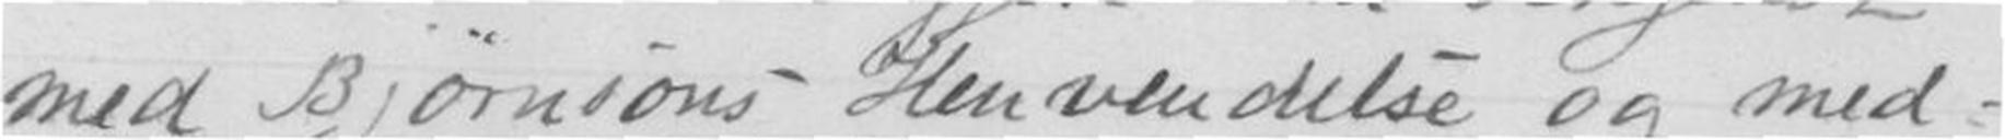med Bjørnsons Henvendelse og med-
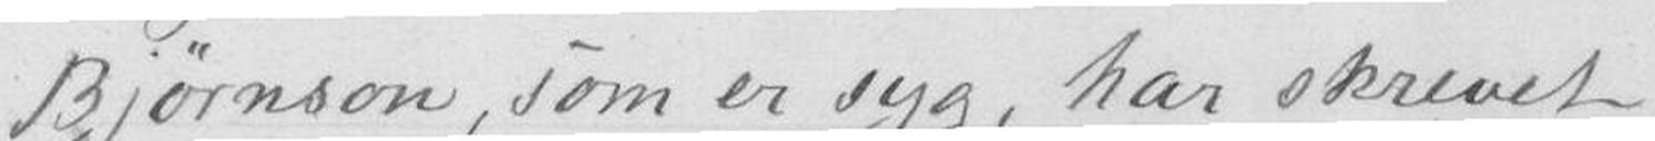Bjørnson, som er syg, har skrevet
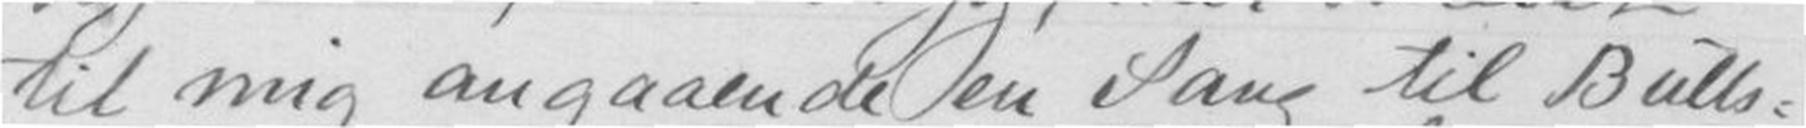til mig angaaende en Sang til Bulls-
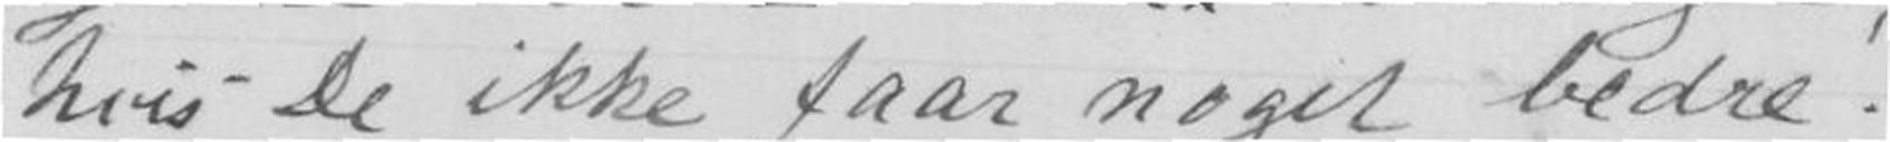hvis De ikke faar noget bedre.
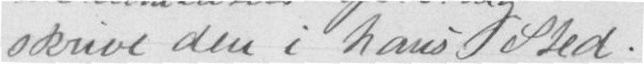skrive den i hans Sted.
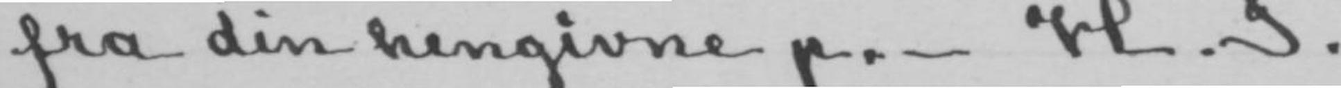fra din hengivne p.- H. I.
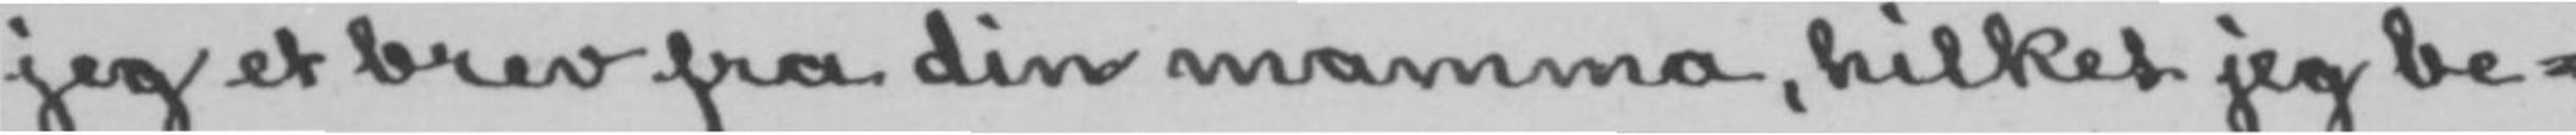jeg et brev fra din mamma, hvilket jeg be-
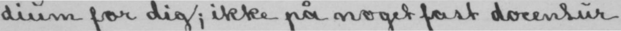dium for dig; ikke på noget fast docentur
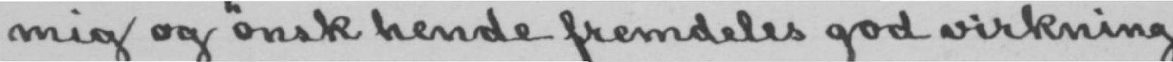mig og ønsk hende fremdeles god virkning
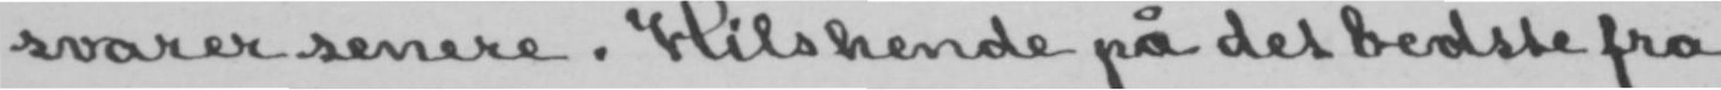svarer senere. Hils hende på det bedste fra
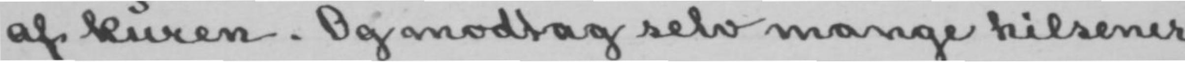af kuren. Og modtag selv mange hilsener
